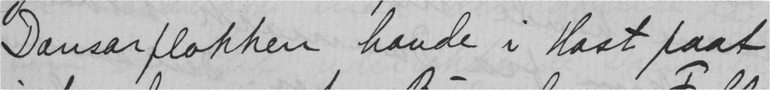Dansarflokken havde i Hast faat
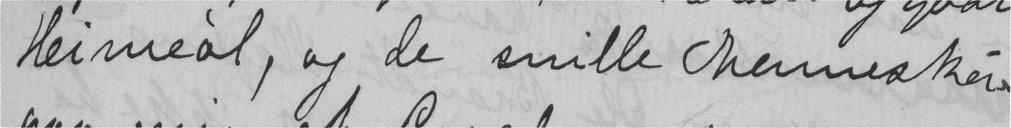Heimeøl, og de snille mennesker
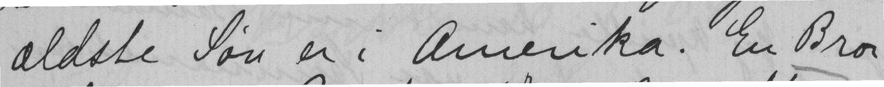ældste Søn er i Amerika. En Bror
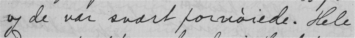og de var svært fornøiede. Hele
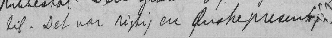til. Det var rigtig en Ønskepresent
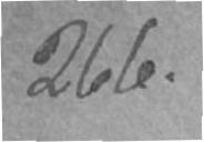266.
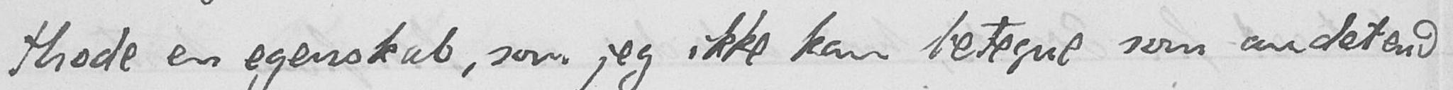thode en egenskab, som jeg ikke kan betegne som andet end
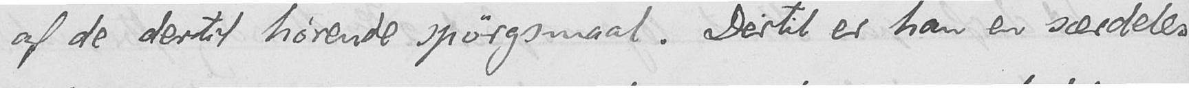af de dertil hørende spørgsmaal. Dertil er han en særdeles
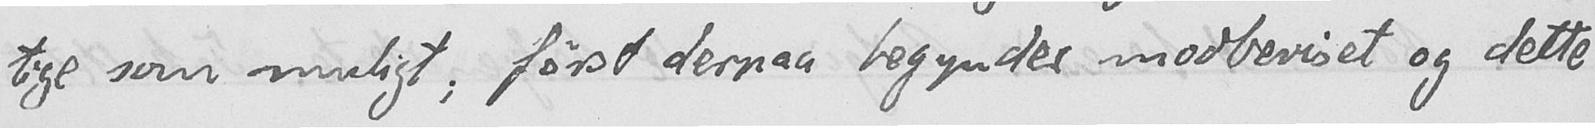tige som muligt; først derpaa begynder modbeviset og dette
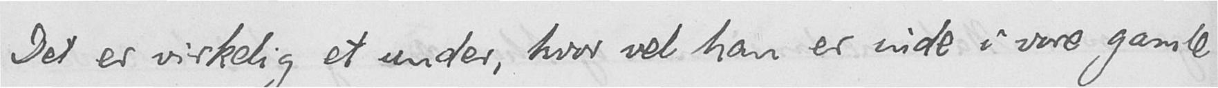Det er virkelig et under, hvor vel han er inde i vore gamle
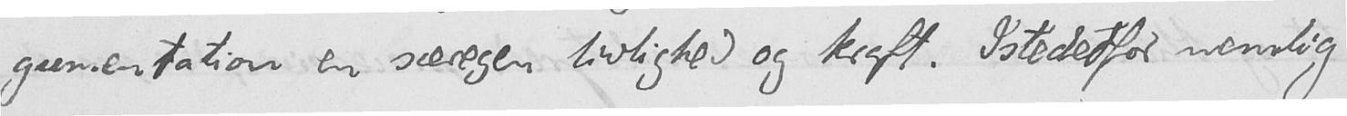gumentation en særegen livlighed og kraft. Istedetfor nemlig
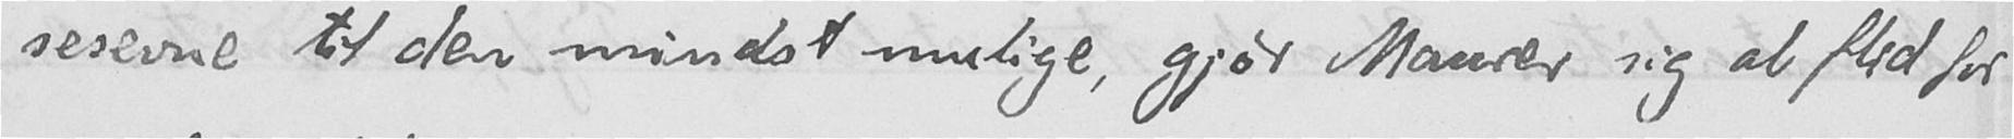sesevne til den mindst mulige, gjør Maurer sig al flid for
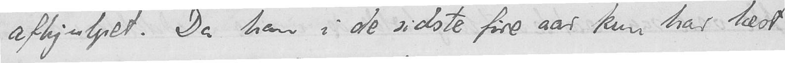afhjulpet. Da han i de sidste fire aar kun har læst
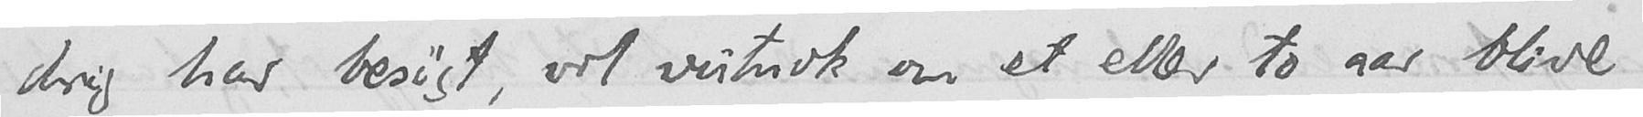drig har besøgt, vil vistnok om et eller to aar blive
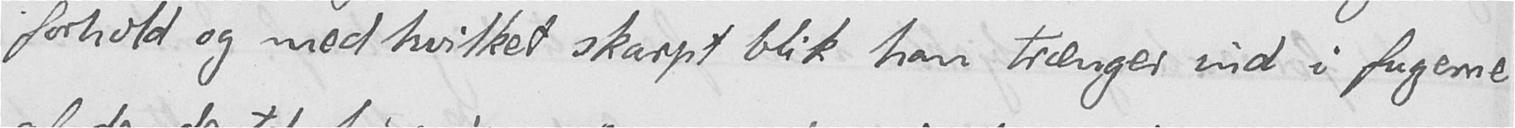forhold og med hvilket skarpt blik han trænger ind i fugerne
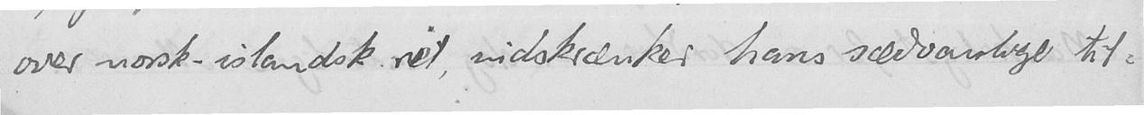over norsk-islandsk ret, indskrænker hans sædvanlige til-
